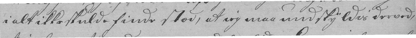i alt ikke skulde finde stæd, at ieg maa undskyldis derved,
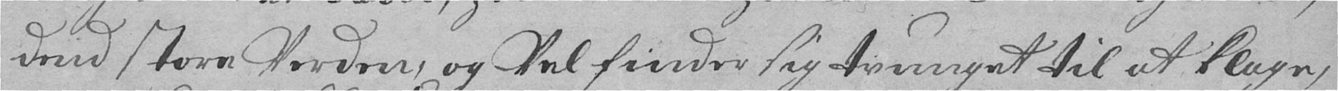dend store Verden, og Vel finder sig tvunget til at klage,
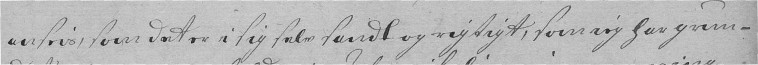anseis, som det er i sig selv sandt og rigtigt, som ieg har grun-
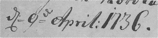d. 9de April 1736.
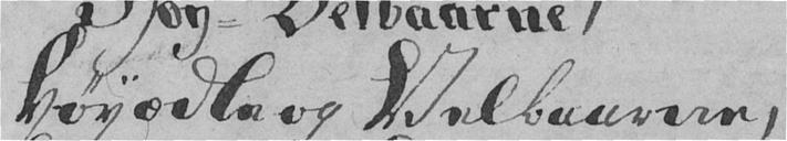Høyædle og Velbaarne,
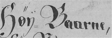Høy-Baarne,
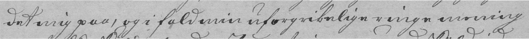det mig paa, og i fald min uforgribelige ringe mening
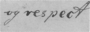og respect
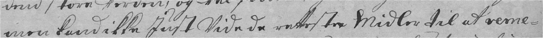men kand ikke Iust Vide de retteste Midler til at reme-
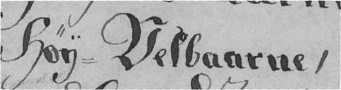Høy-Velbaarne,
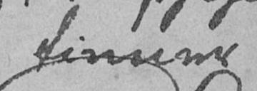tinsem
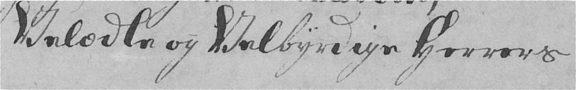Velædle og Velbyrdige Herrers
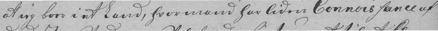at ieg boer i et Land, hvor mand har liden Connoissance af
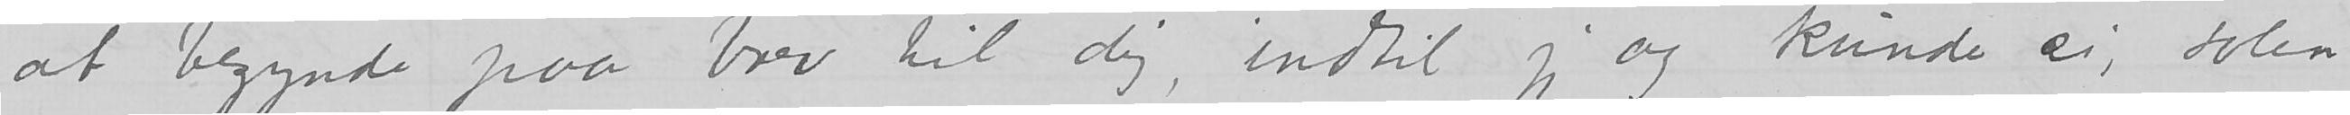at begynde paa brev til dig, indtil jeg og kunde si, solen
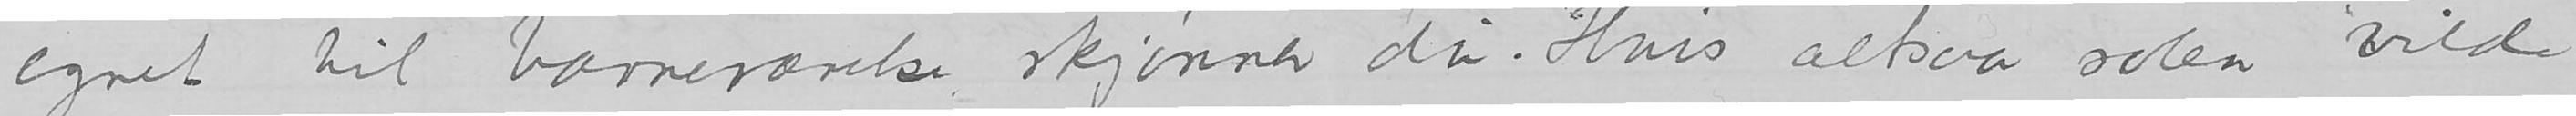egnet til barneværelse skjønner du. Hvis altsaa solen vilde
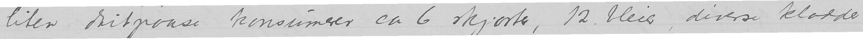liten dritpaase konsumerer ca 6 skjorter, 12 bleier, diverse kladder
